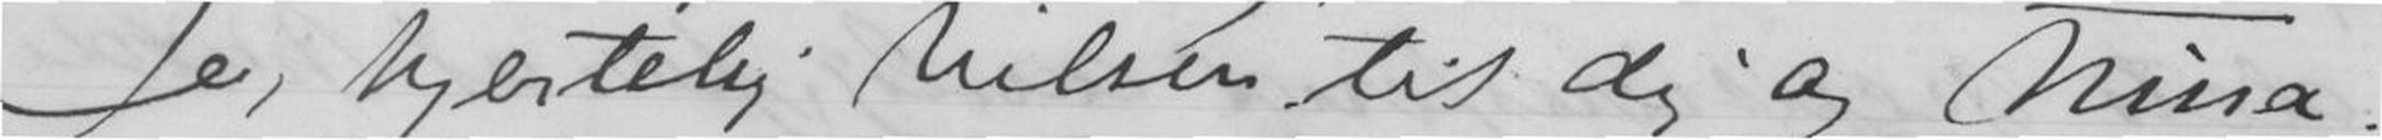Se, hjertelig hilsen til dig og Nina!
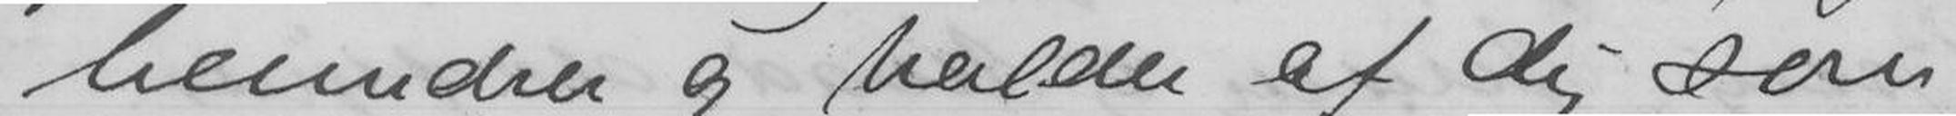beundrer og holder af dig som
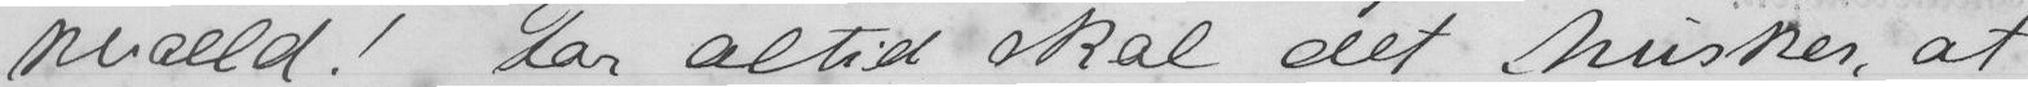kvæld! For altid skal det huskes, at
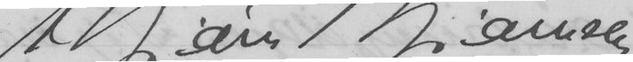Bjørn Bjørnson
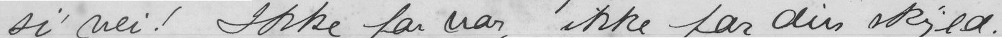si nei! Ikke for vor, ikke for din skyld!
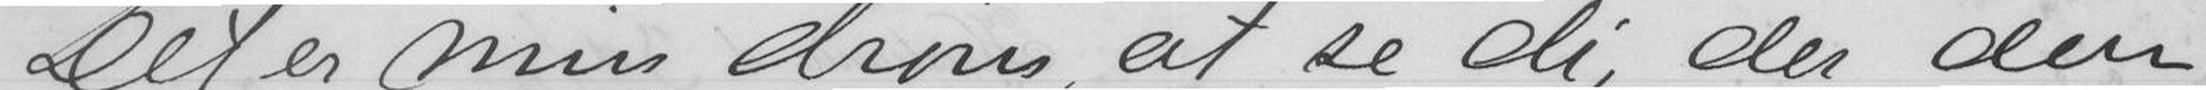Det er min drøm, at se dig der den
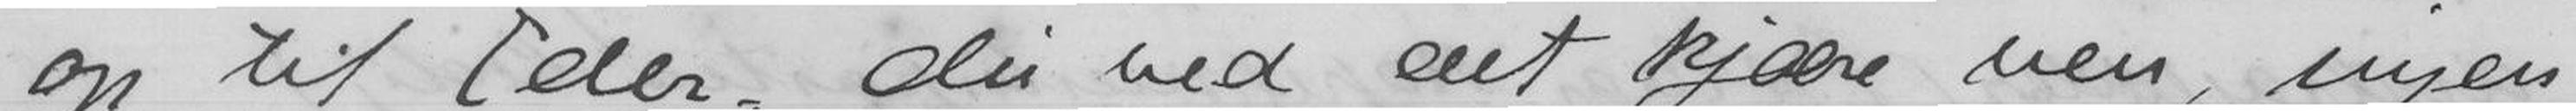op til Eder og du ved det, kjære ven, ingen
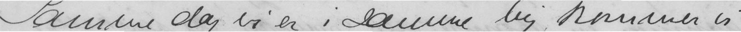Samme dag vi er i sammen by, kommer vi
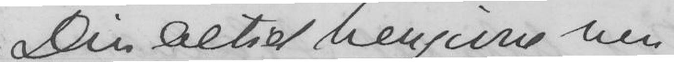Din altid hengivne ven
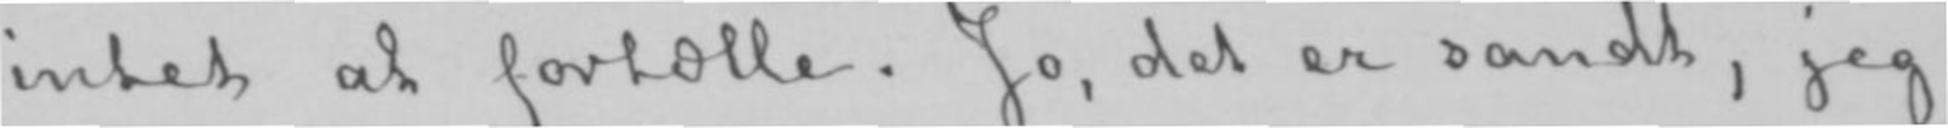intet at fortælle. Jo, det er sandt, jeg
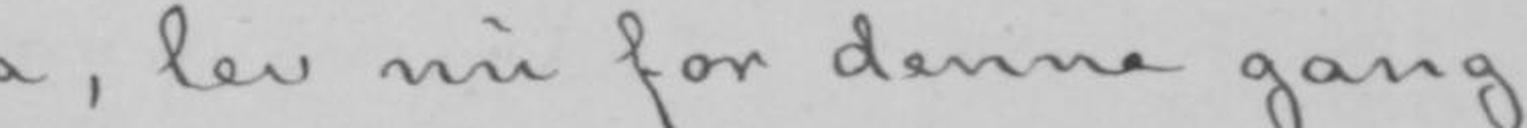Ja, lev nu for denne gang
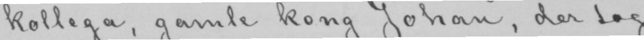kollega, gamle kong Johan, der tog
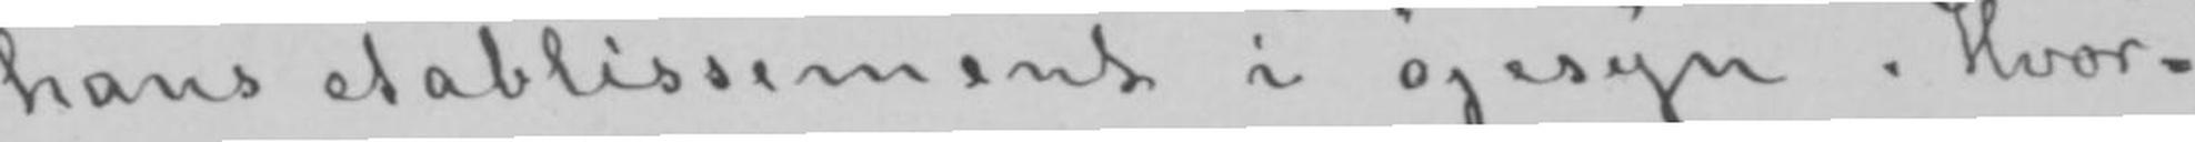hans etablissement i øjesyn. Hvor-
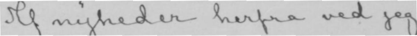Af nyheder herfra ved jeg
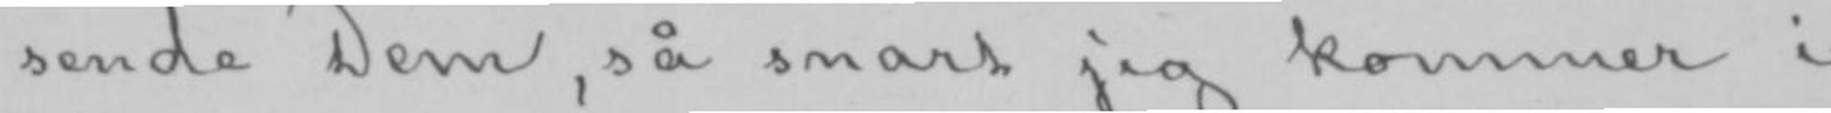sende Dem, så snart jeg kommer i
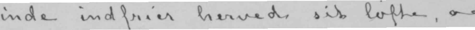inde indfrier herved sit løfte, og
